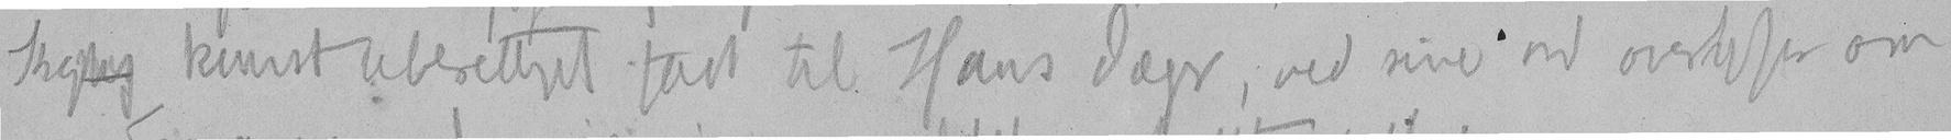Ihgting kunst uberettiget fast til Hans Jæger, ved sine ord overlefer om
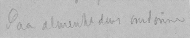Paa almenhedens omdømme
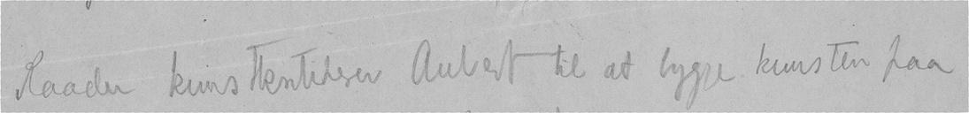Raader kunstkritikeren Aubert til at bygge kunsten paa
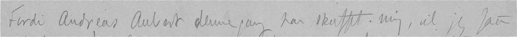Fordi Andreas Aubert denne gang har skuffet mig, vil jeg faa
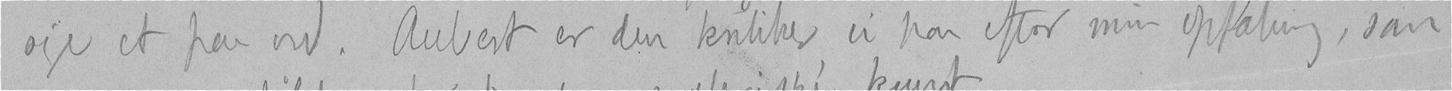sige et par ord. Aubert er den kritiker vi har efter min opfating, som
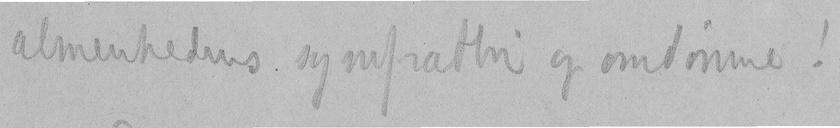almenhedens sympathi og omdømme.
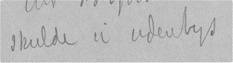skulde vi udenbys
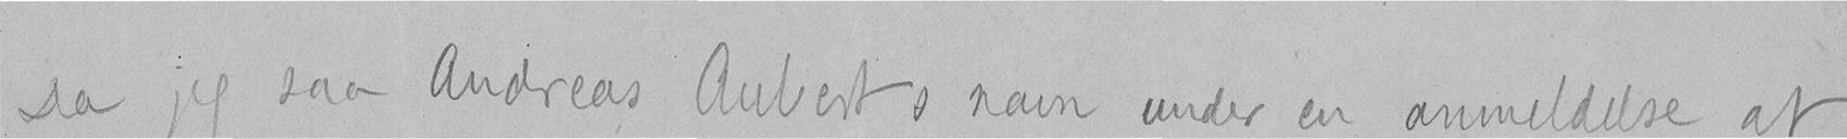Da jeg saa Andreas Auberts navn under en anmeldelse af
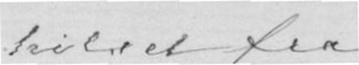hilset fra
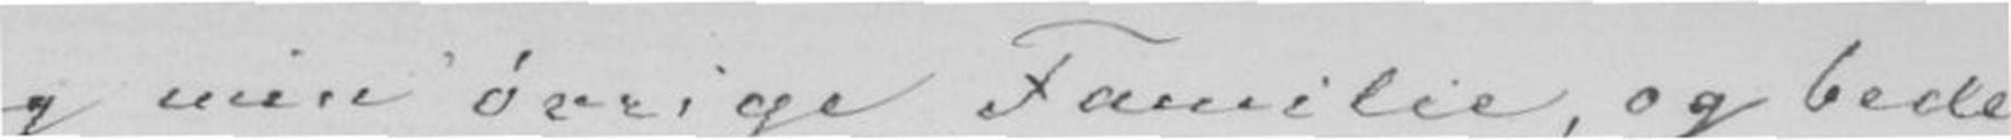og min øvrige Familie, og beder
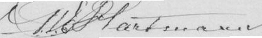J. P. E. Hartmann
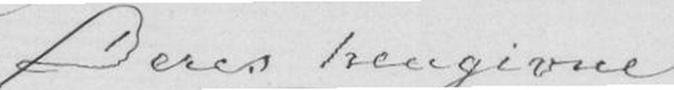Deres hengivne
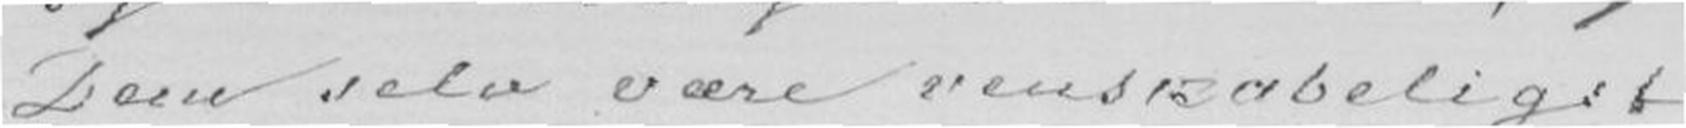Dem selv være venskabeligst
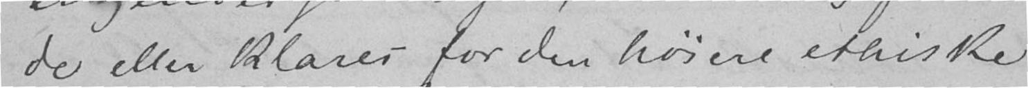de eller klares for den høiere ethiske
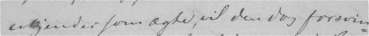erkjendes som ægte, vil den dog forsvin-
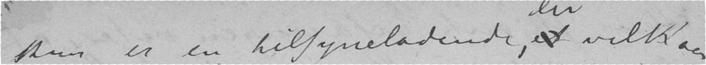kun er en tilsyneladende vilkaar-
